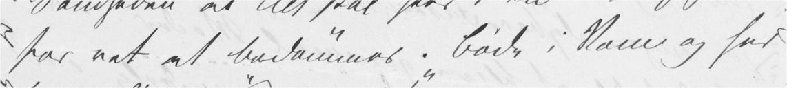for ret at bedømmes. Både i Rom og her
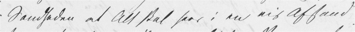Sandheden at Alt skal sees i en vis Afstand
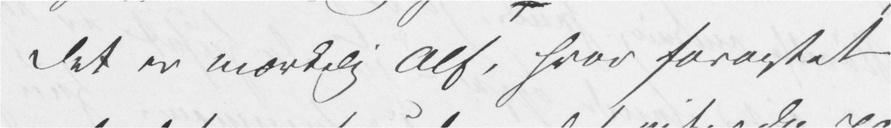Det er mærkelig Alf, hvor foragtet
